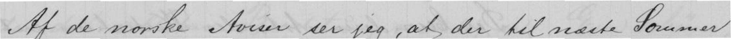Af de norske Aviser ser jeg, at der til næste Sommer
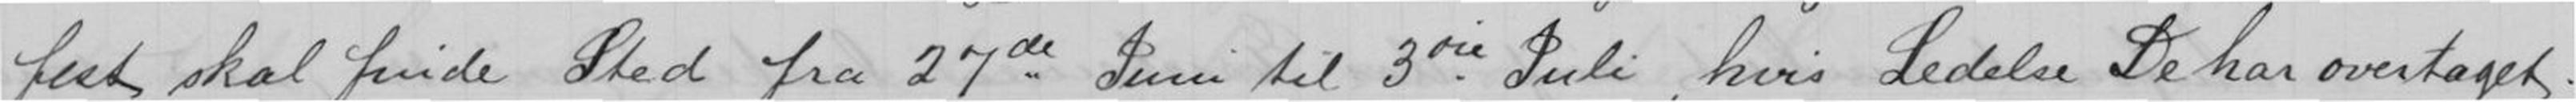fest skal finde Sted fra 27de Juni til 3die Juli, hvis Ledelse De har overtaget.
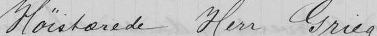Høistærede Herr Grieg!
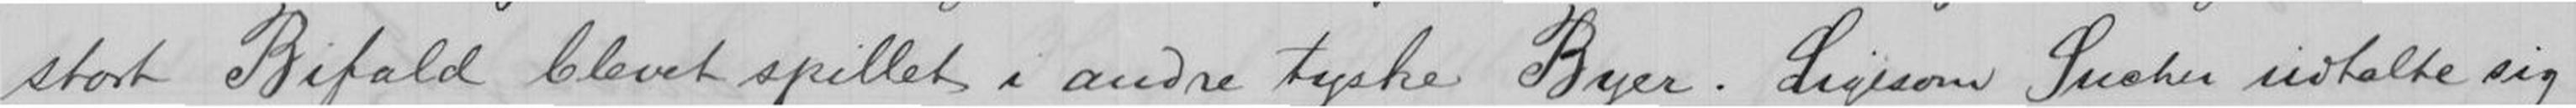stort Bifald blevet spillet i andre tyske Byer. Ligesom Sucher udtalte sig
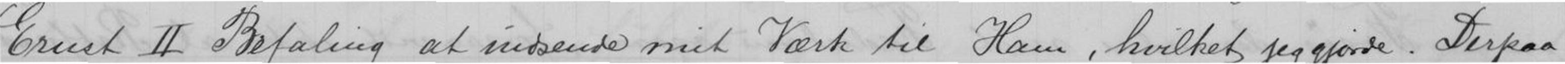Ernst II Befaling at indsende mit Værk til Ham, hvilket jeg gjorde. Derpaa
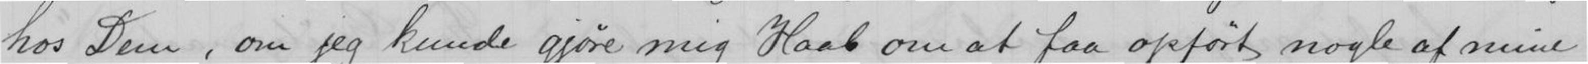hos Dem, om jeg kunde gjøre mig Haab om at faa nogle af mine
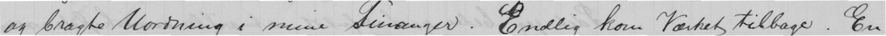og bragte Uordning i mine Tinanger. Endlig kom Værket tilbage. En
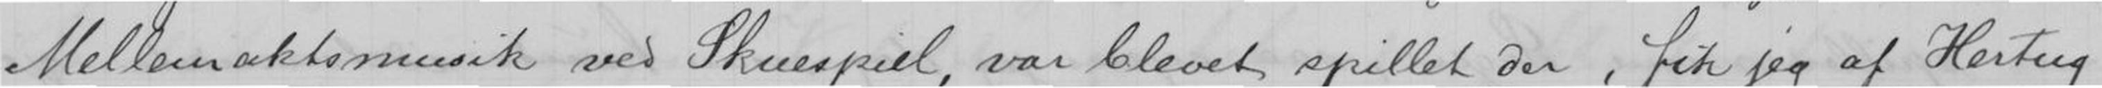Mellemaktsmusik ved Skuespill, var blevet spillet der, fik jeg af Hertug
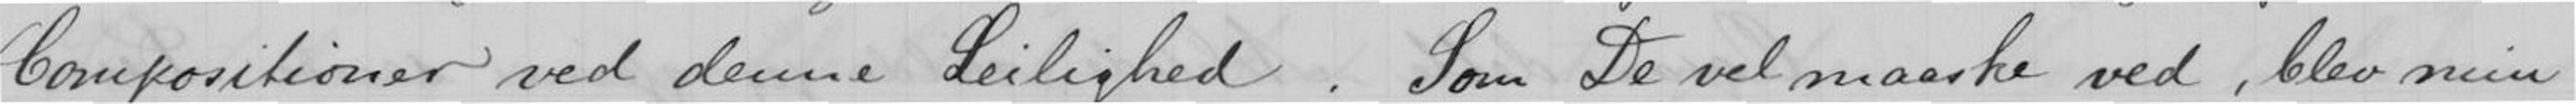Composioner ved denne Leilighed. Som De vel maaske ved, blev min
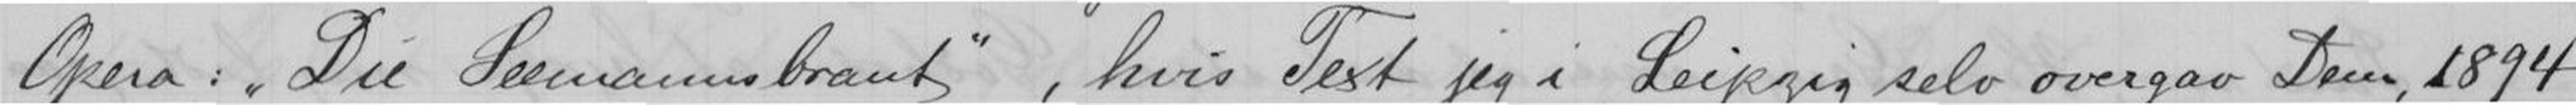Opera: Du Seemannsbraut, hvis Text jeg i Leipzig selv overgav Dem, 1894
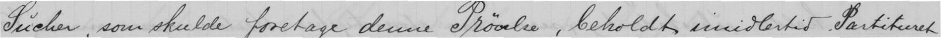Sucher, som skulde foretage denne Prøvelse, beholdt imidlertid Partituret
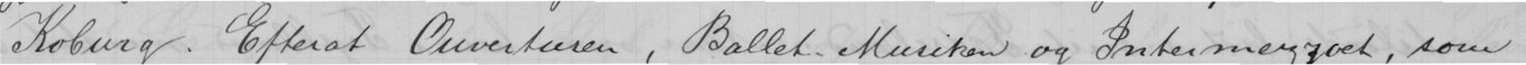Koburg. Efterat Ouverturen, Ballet Musiken og Intermezzoet, som
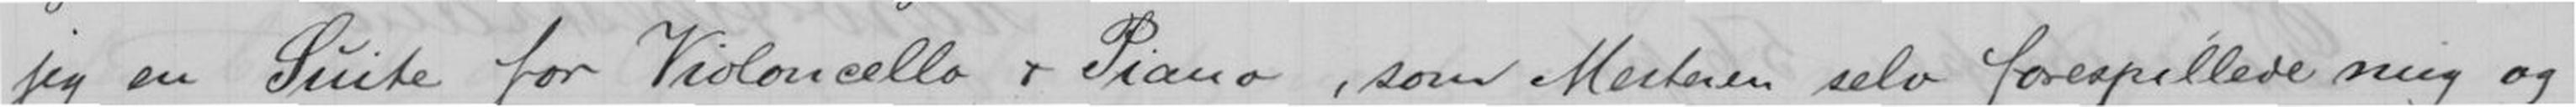jeg en Suite for Violincello, Piano, som Mesteren selv forespillede mig og
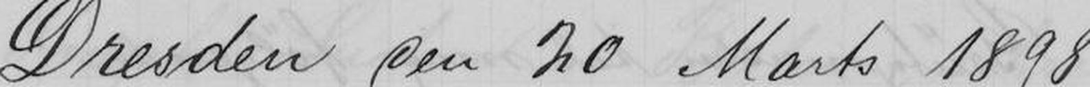Dresden den 20 Marts 1898
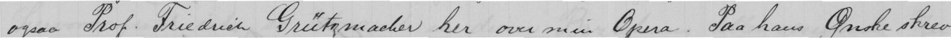ogsaa Prof. Friedrich Grützmacher her over min Opera. Paa hans Ønske skrev
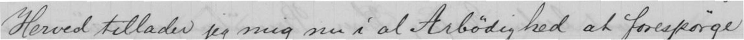Herved tillader jeg mig nu i al Ærbødighed at forespørge
